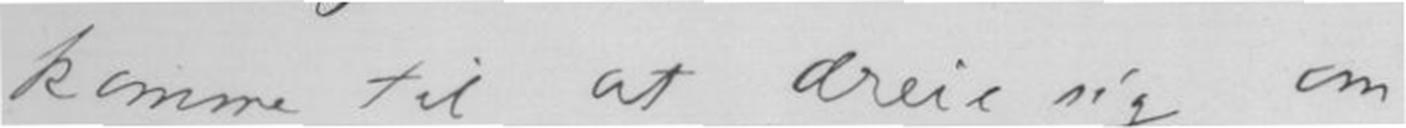komme til at dreie sig om
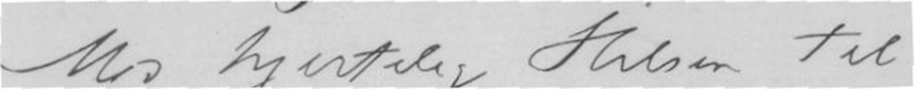Med hjertelig Hilsen til
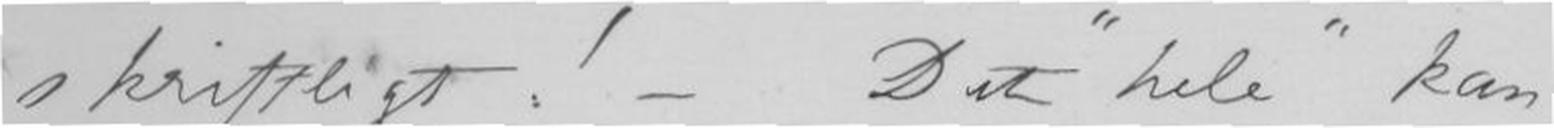skriftligt! - Det "hele" kan
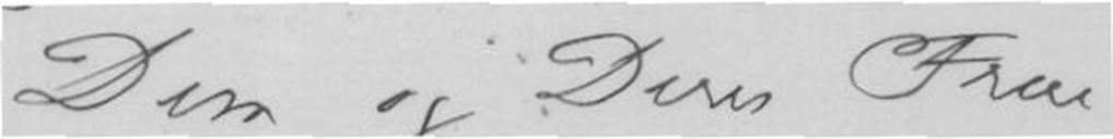Dem og Deres Frue
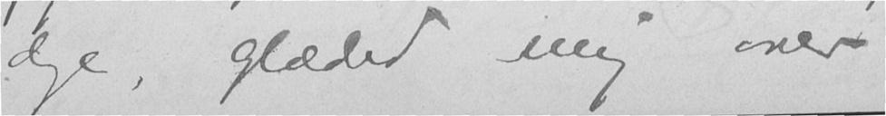dage, glæded mig over
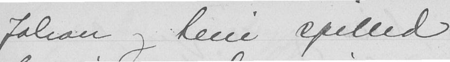Johan og Leni spilled
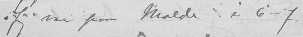Jeg var paa Molde i 6- 7
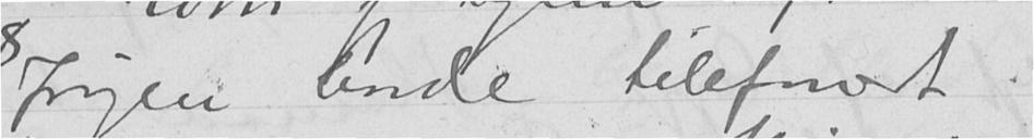Jørgen havde telefonert
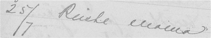25/7 Reiste mama
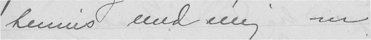tennis med mig om
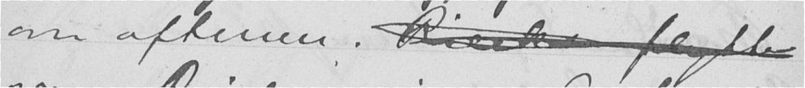om aftenen Riecks flytte
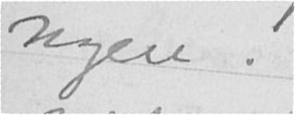nogen.
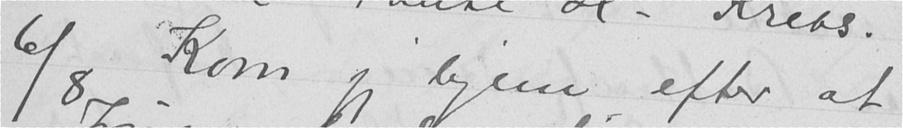6/8 Kom jeg hjem efter at
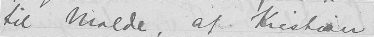til Molde, at Kristian
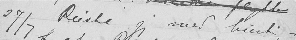27/7 Reiste jeg med hurti-
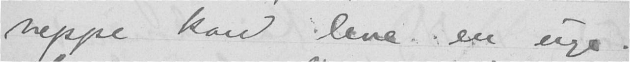neppe kan leve en uge.
